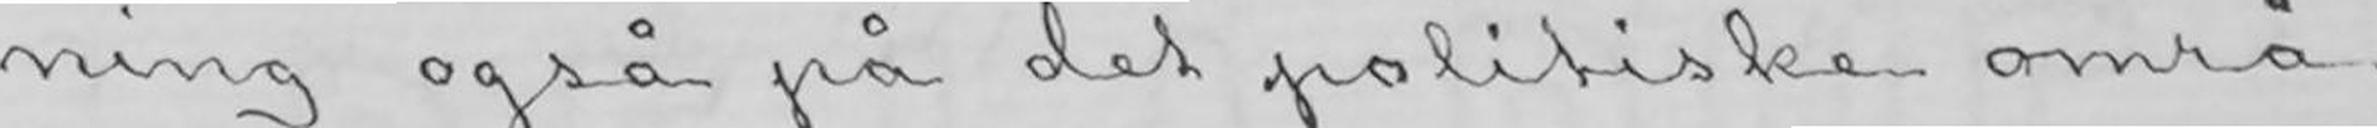ning også på det politiske områ-
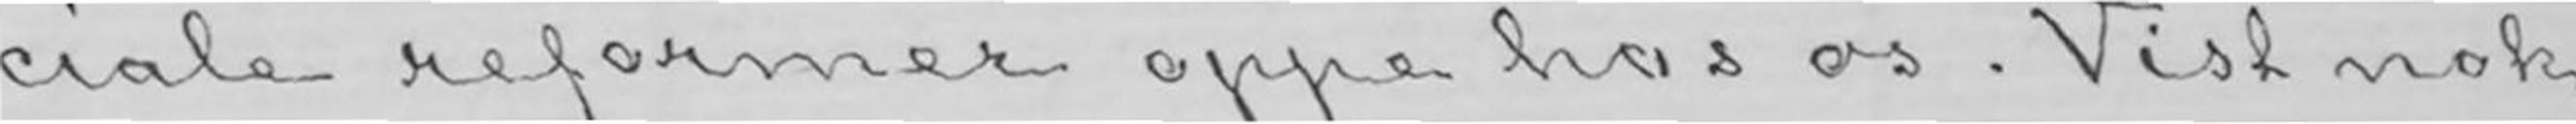ciale reformer oppe hos os. Vist nok
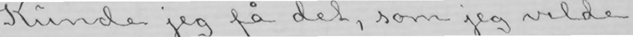Kunde jeg få det, som jeg vilde
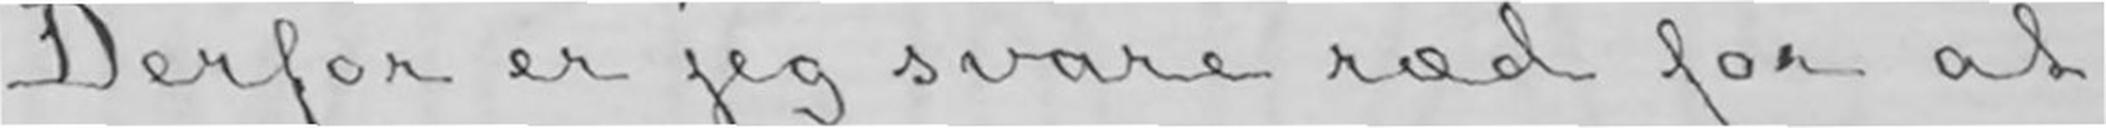Derfor er jeg svare ræd for at
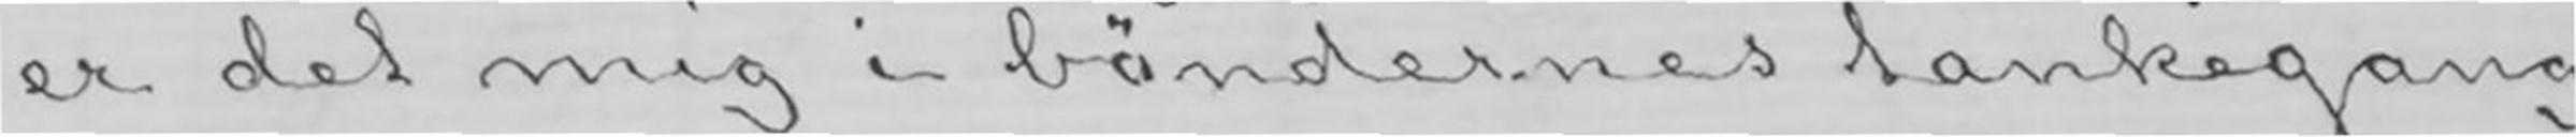er det mig i bøndernes tankegang
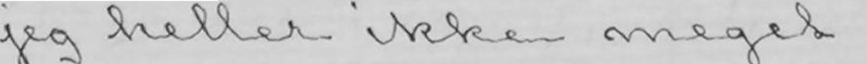jeg heller ikke meget.
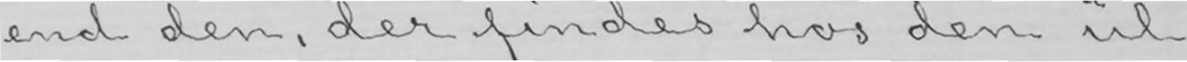end den, der findes hos den ul-
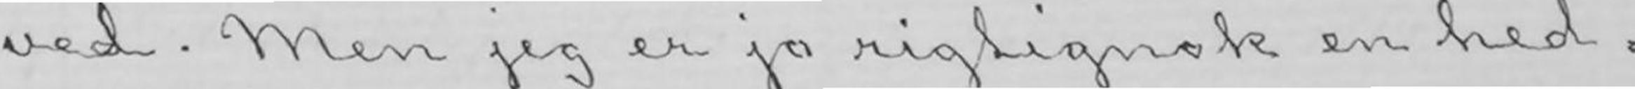ved. Men jeg er jo rigtignok en hed-
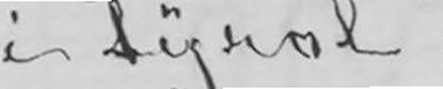i Tyrol.
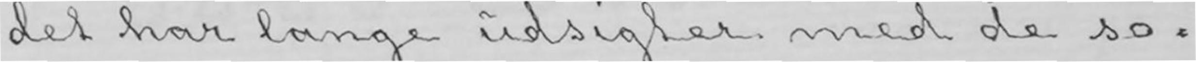det har lange udsigter med de so-
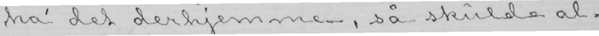ha&rapo; det derhjemme, så skulde al-
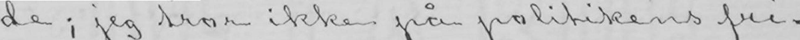de; jeg tror ikke på politikens fri-
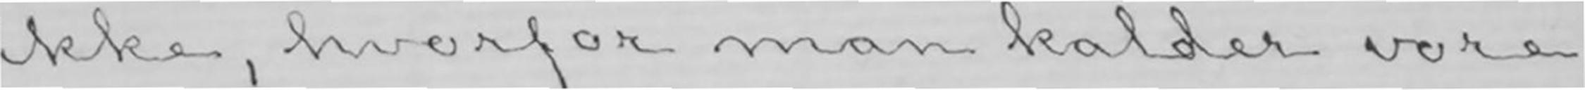ikke, hvorfor man kalder vore
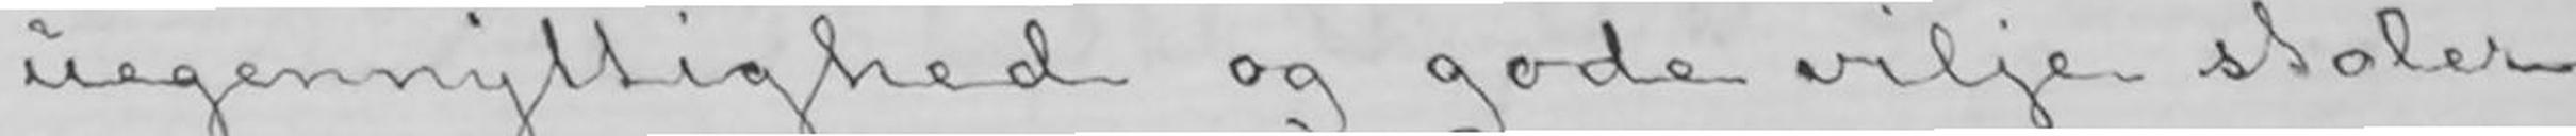uegennyttighed og gode vilje stoler
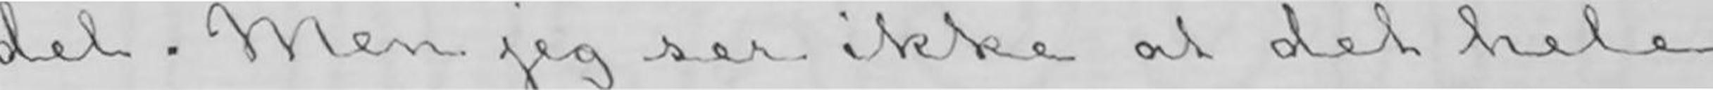del. Men jeg ser ikke at det hele
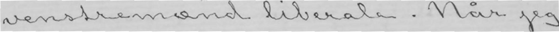venstremænd liberale. Når jeg
